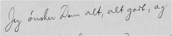Jeg ønsker Dem alt, alt godt, og
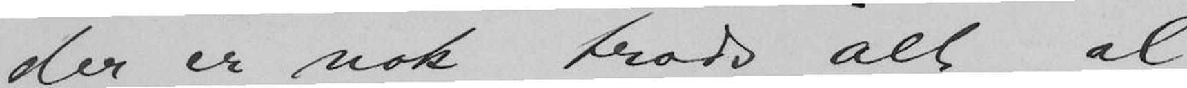der er nok trods alt al
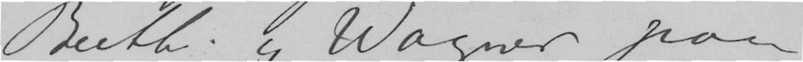Beeth. og Wagner paa
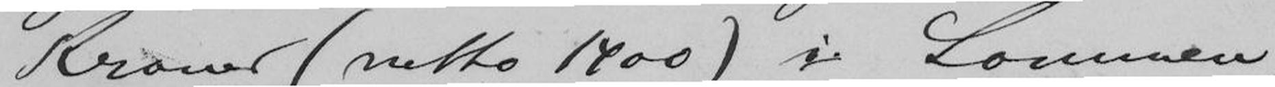Kroner (netto 1400) i Lommen,
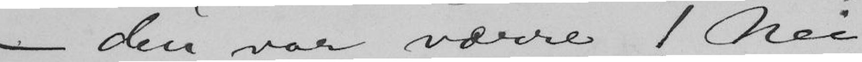- den var værre! Nei
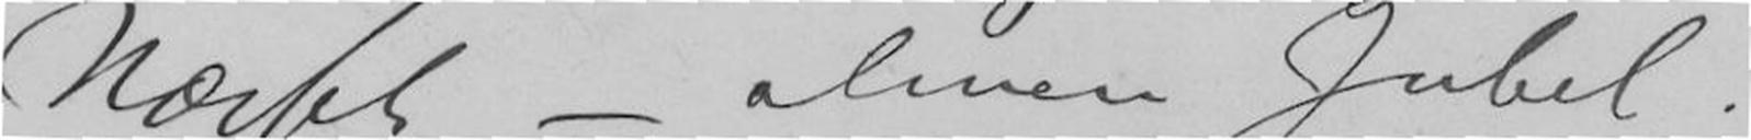Næsset - almen Jubel!
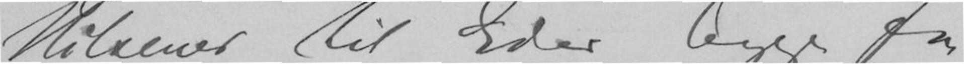Hilsener til Eder begge fra
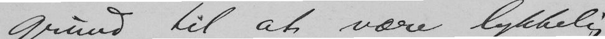Grund til at være lykkelig
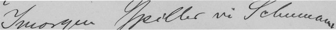Imorgen spiller vi Schumann,
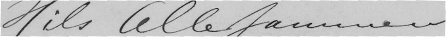Hils Allesammen!
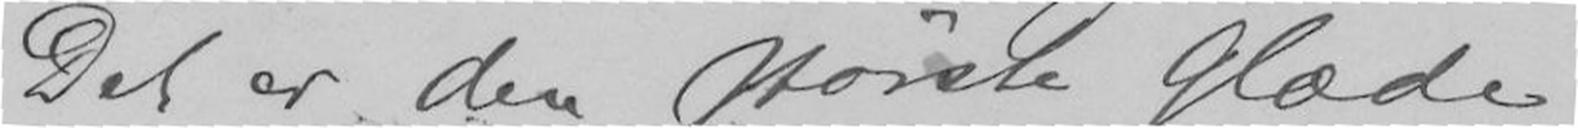Det er den største glæde
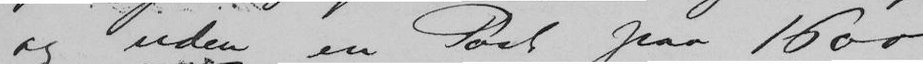og uden en Post paa 1600
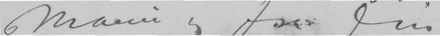Marie og fra Din
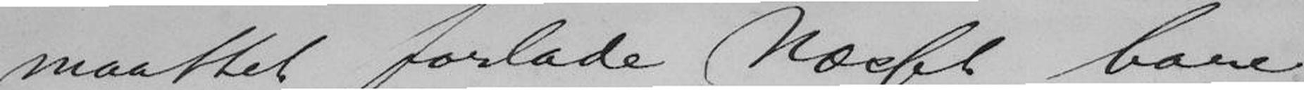maattet forlade Næsset bare
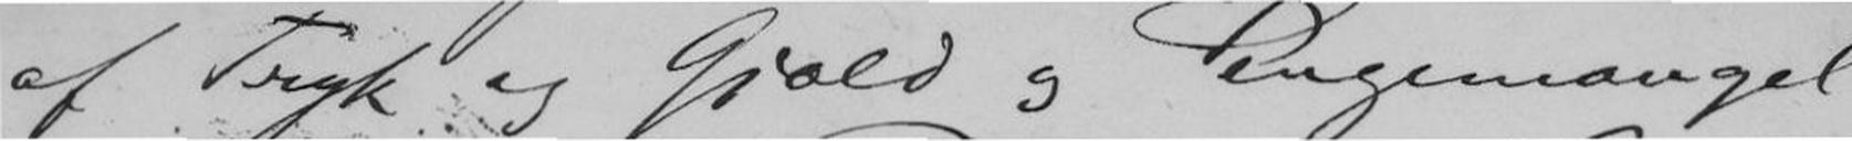af Tryk og Gjæld og Pengemangel
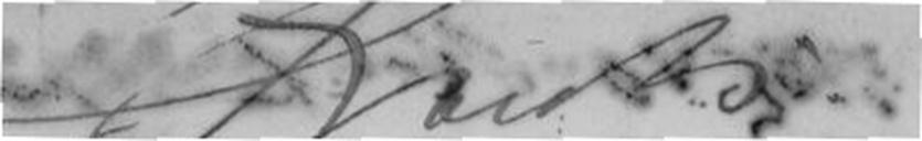Frants
